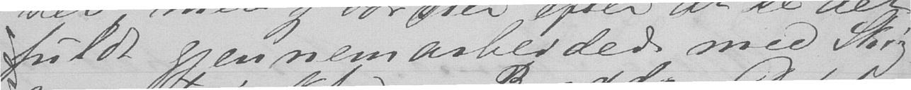fuldt gjennemarbeidede med Skiz-
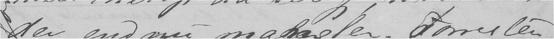der endnu mangler. Forresten
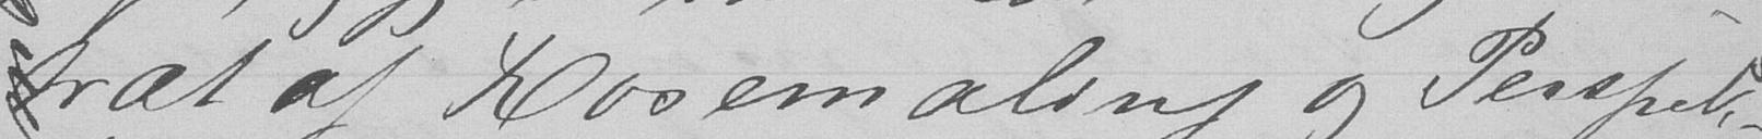træt af Rosemaling og Perspek-
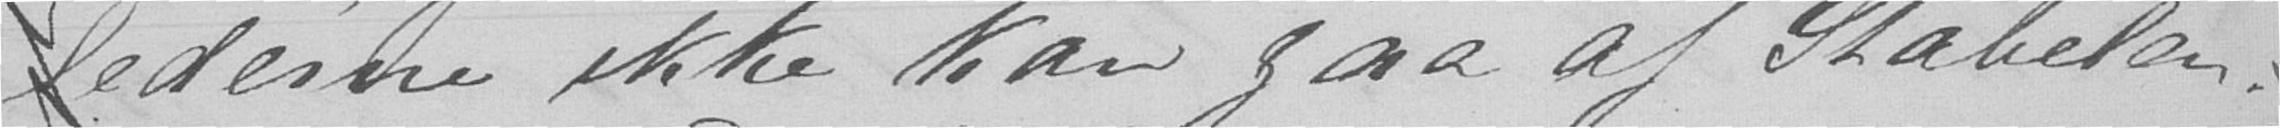lederne ikke kan gaa af Stabelen.
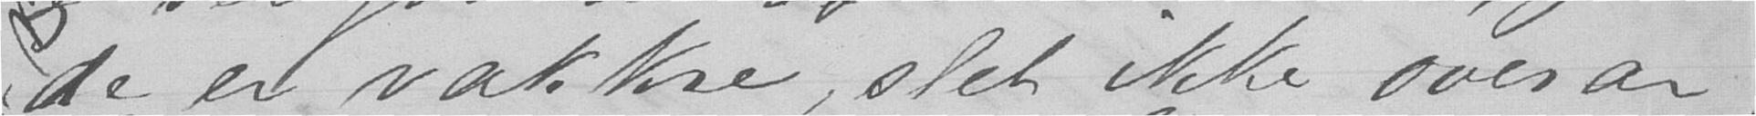de er vakkre, slet ikke overar-
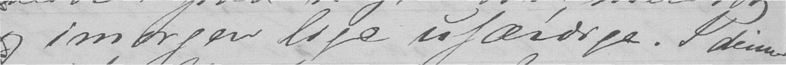og imorgen lige ufærdige. I denne
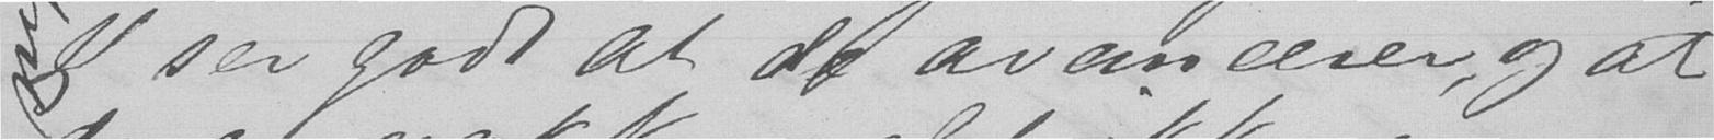Jeg ser godt at de avancerer, og at
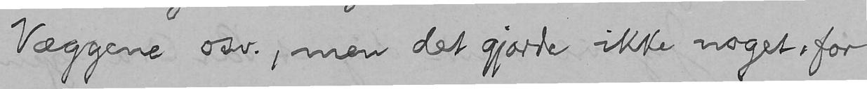Væggene osv., men det gjorde ikke noget, for
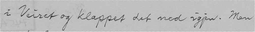i Veiret og klappet det ned igjen. Men
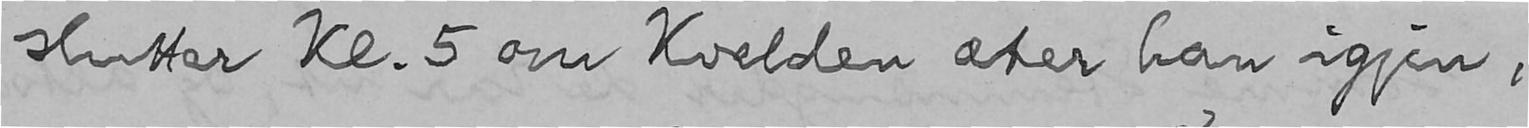slutter Kl. 5 om Kvelden æter han igjen,
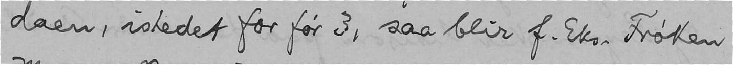daen, istedet for før 3, saa blir f.Eks. Frøken
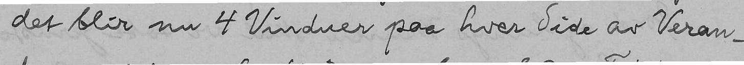det blir nu 4 Vinduer paa hver Side av Veran-
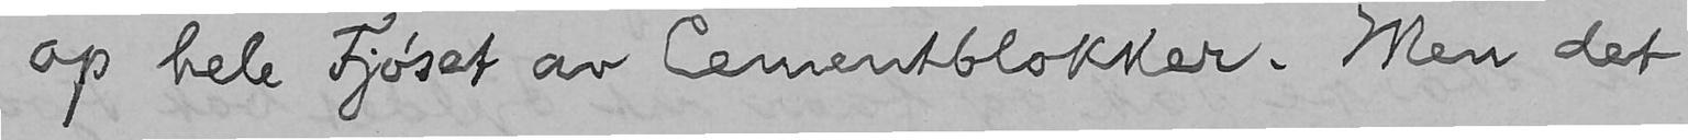op hele Fjøset av Cementblokker. Men det
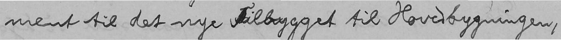ment til det nye Tilbygget til Hovedbygningen,
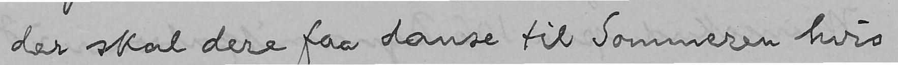der skal dere faa danse til Sommeren hvis
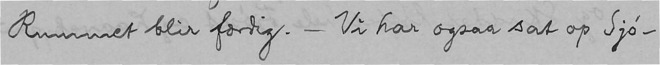Rummet blir færdig. - Vi har ogsaa sat op Sjø-
